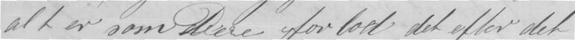alt er som Dere forlod det efter det
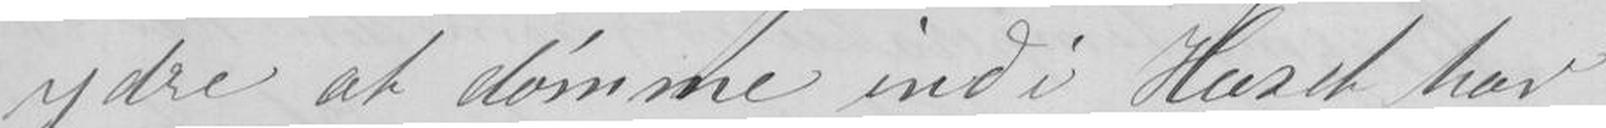ydre at dømme ind i Huset har
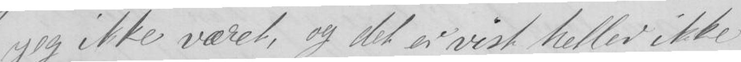jeg ikke været, og det er vist heller ikke
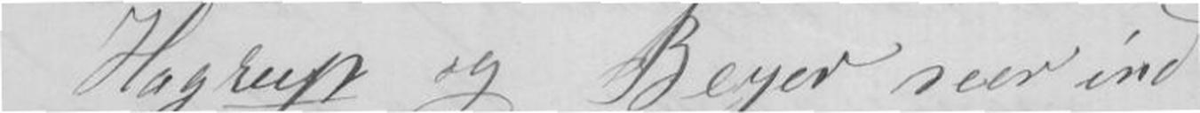Hagerup og Beyer seer ind
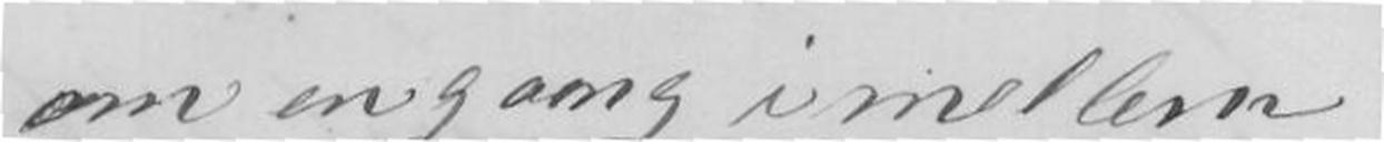om engang imellem -
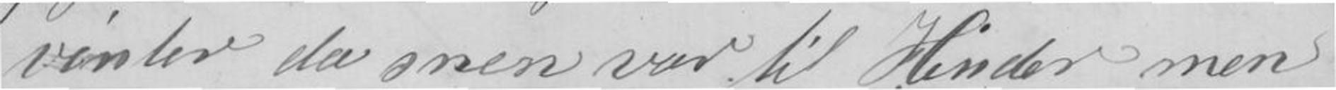vinter da snen var til Hinder men
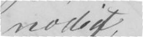nødigt.
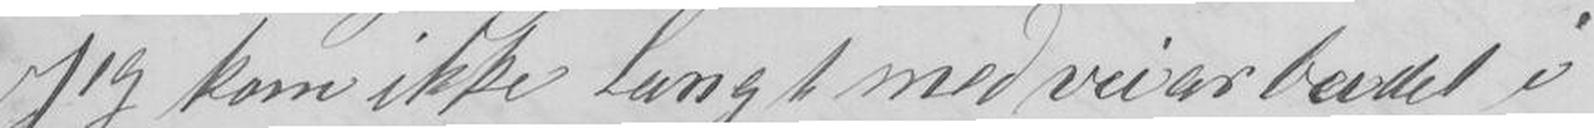Jeg kom ikke langt med veiarbeidet i
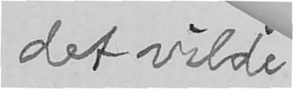det vilde
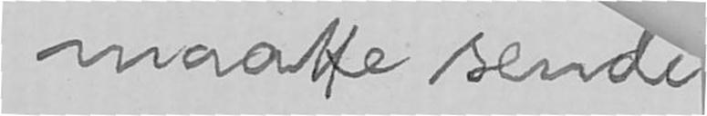maate sende
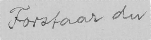Forstaar du
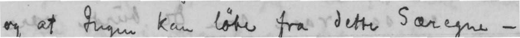og at Ingen kan løbe fra dette Særegne -
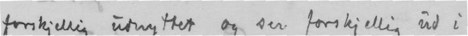forskjellig udnyttet og ser forskjellig ud i
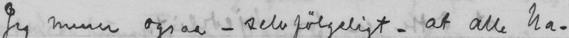Jeg mener ogsaa - selvfølgeligt - at alle Na-
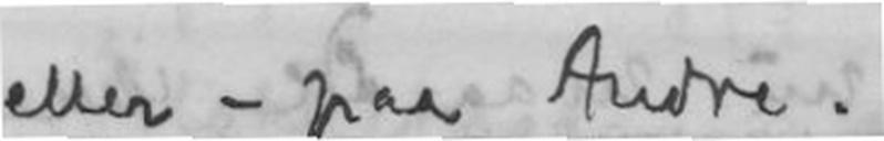eller - paa Andre.
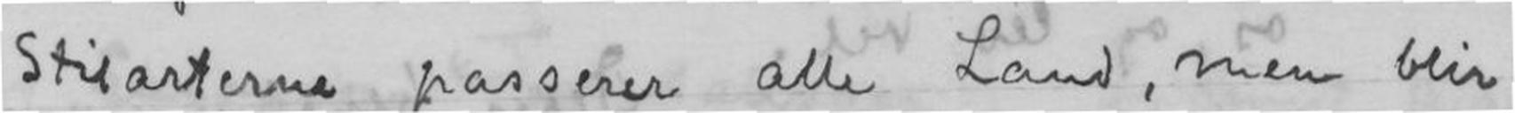Stilarterne passerer alle Land, men blir
